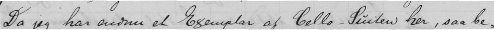Da jeg har endnu et Exemplar af Cello-Suiten her, saa be-
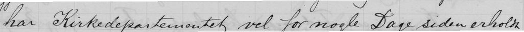har Kirkedepartementet vel for nogle Dage siden erholdt,
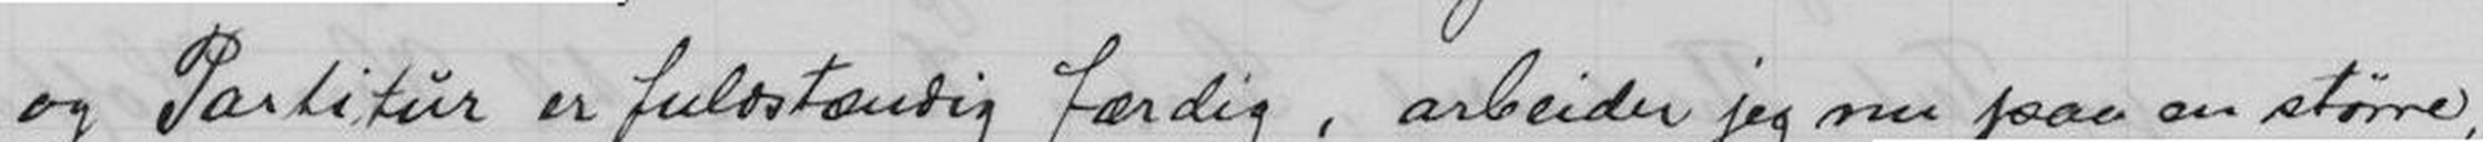og Partitur er fuldstændig færdig, arbeider jeg nu paa en større
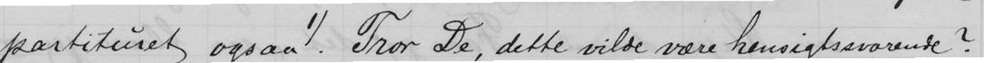partituret ogsaa 1) Tror De, dette vilde være hensigsvarende?
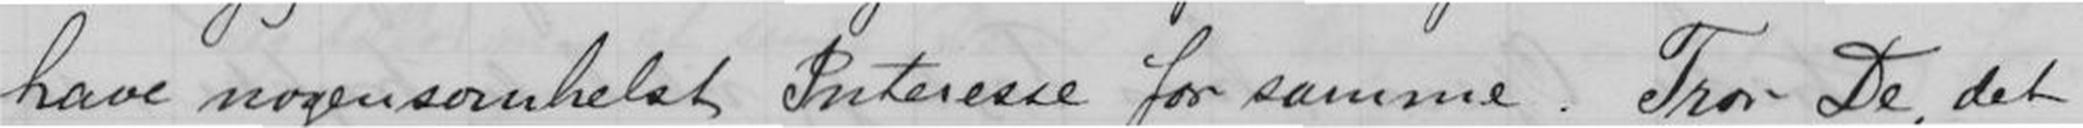have nogensomhelst Interesse for samme. Tror De, det
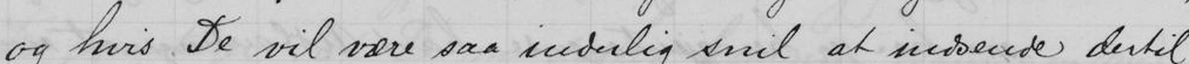og hvis De vil være saa inderlig snil at indsende dertil
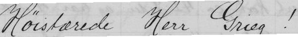Høistærede Her Grieg!
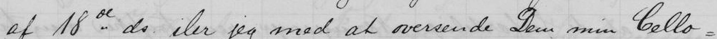af 18de ds iler jeg med at oversende Dem Cello-
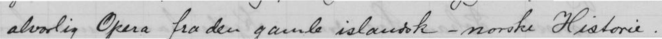alvorlig Opera fra gamle islandsk- norske Historie.
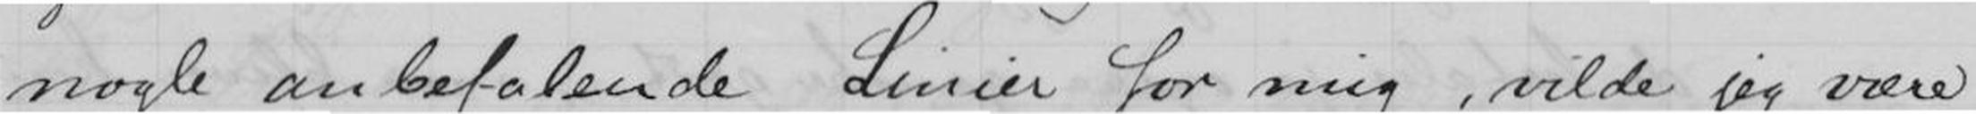nogle anbefalende Linier for mig, vilde jeg være
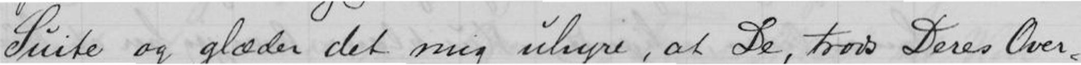Suite og glæder det mig uhyre, at De, trods Deres Over-
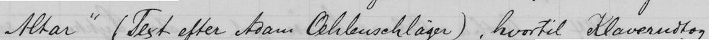Altar" (Text efter Adam Oehlenschläger), hertil Klaverudtag
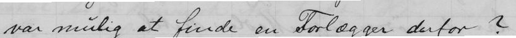var mulig at finde en Forlægger derfor? -
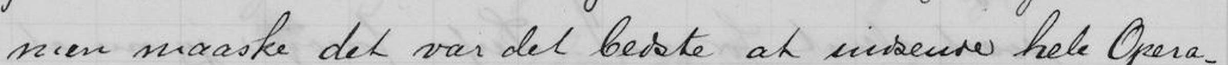men maaske det var det bedste at indsende hele Opera-
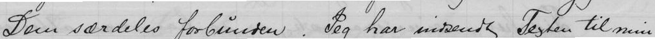Dem særdeles forbunden. Jeg har indsendt Texten til min
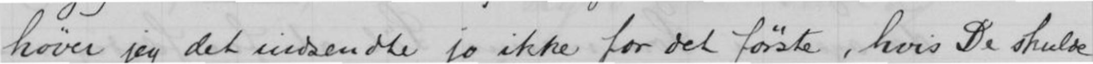høver jeg det indsendte jo ikke for det første, hvis De skulde
